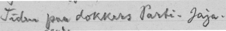Tiden paa dokkers Parti. Jaja.
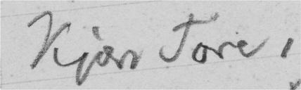Kjære Tore,
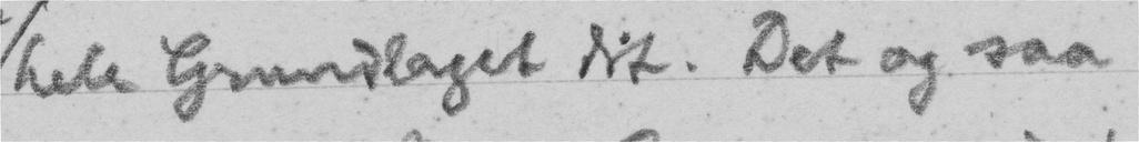hele Grundlaget dit. Det og saa
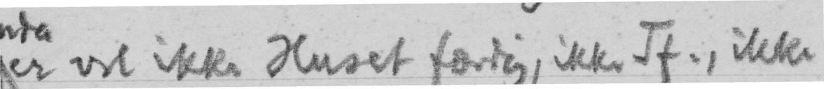er vel ikke Huset færdig, ikke Tf., ikke
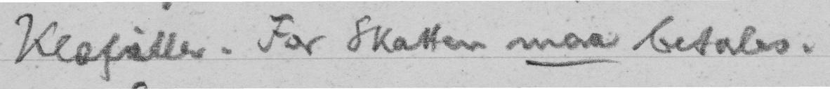Klæfiller. For Skatten maa betales.
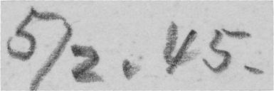5/2. 45.
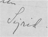Sigrid
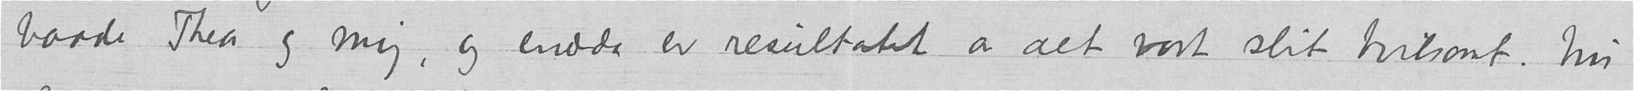baade Thea og mig, og endda er resultatet av alt vort slit tvilsomt. Nu
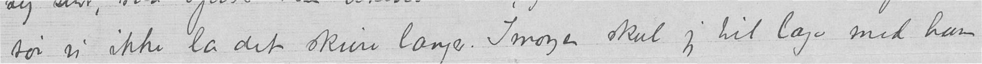tør vi ikke la det skure længer. Imorgen skal jeg til læge med ham
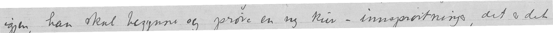igjen, han skal begynne og prøve en ny kur – innsprøitninger, det er det
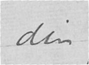din
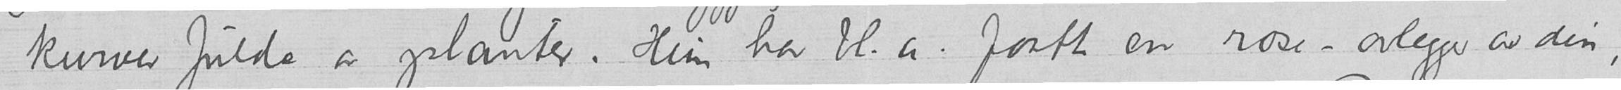kurver fulde av planter. Hun har bl.a. faatt en rose-avlegger av din,
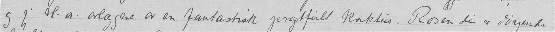og jeg bl.a. avlæggere av en fantastisk pragtfull kaktus. Rosen din er dørgende
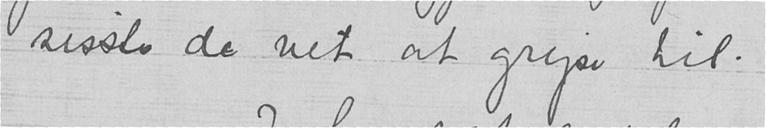sisste de vet at gripe til.
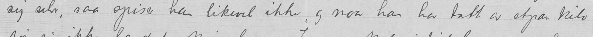sig selv, saa spiser han likevel ikke, og naar han har tatt av etpar kilo
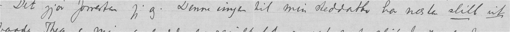- Det gjør forresten jeg og. Denne ungen til min steddatter har næsten slitt ut
